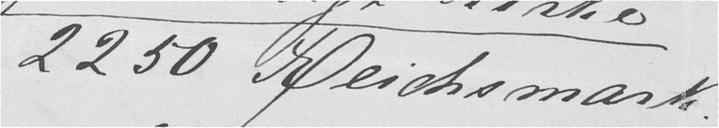2250 Reichsmark.
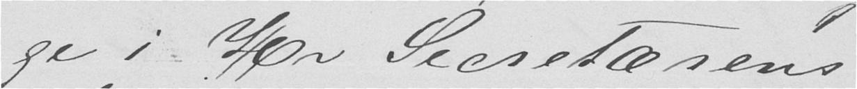ge i Hr Secretærens
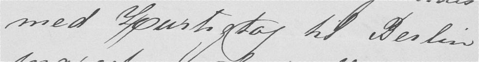med Hurtigtog til Berlin
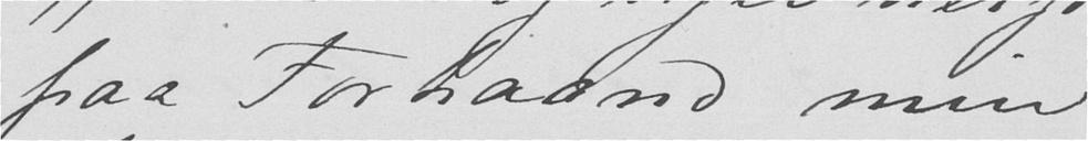paa Forhaand min
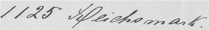1125 Reichsmark.
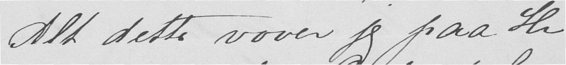Alt dette vover jeg paa Hr
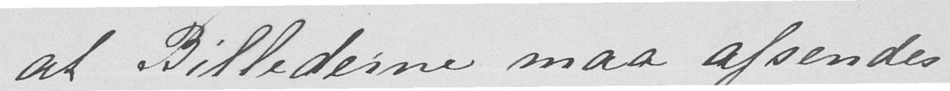at Billederne maa afsendes
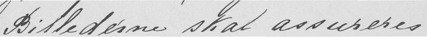Billederne skal assureres
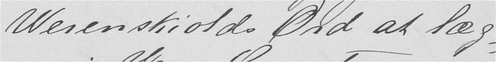Werenskiolds Ord at læg-
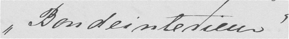"Bondeinterieur"
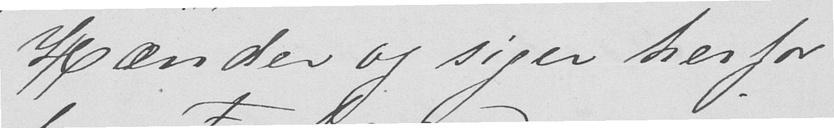Hænder og siger herfor
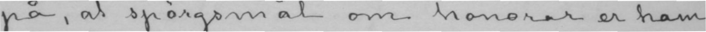på, at spørgsmål om honorar er ham
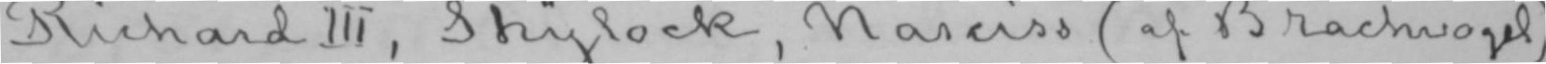Richard III, Shylock, Narciss (af Brachvogel)
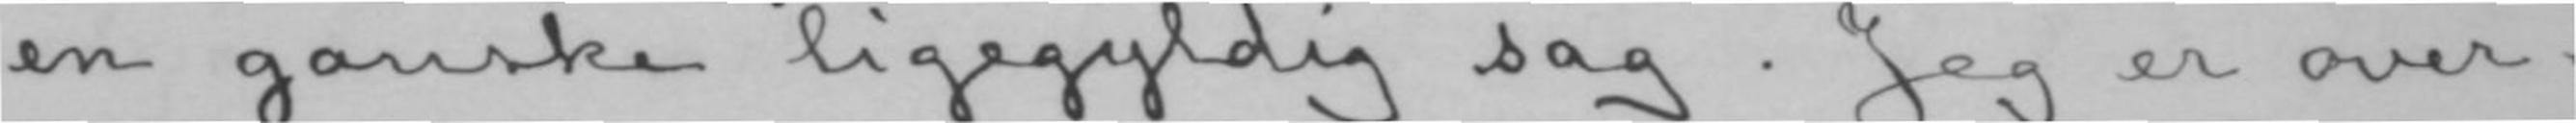en ganske ligegyldig sag. Jeg er over-
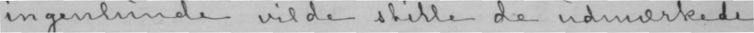ingenlunde vilde stille de udmærkede
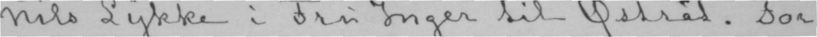Nils Lykke i Fru Inger til Østråt. For
